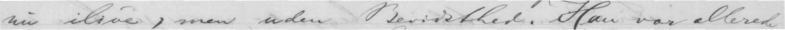nu ilive, men uden Bevidsthed. Han var allerede
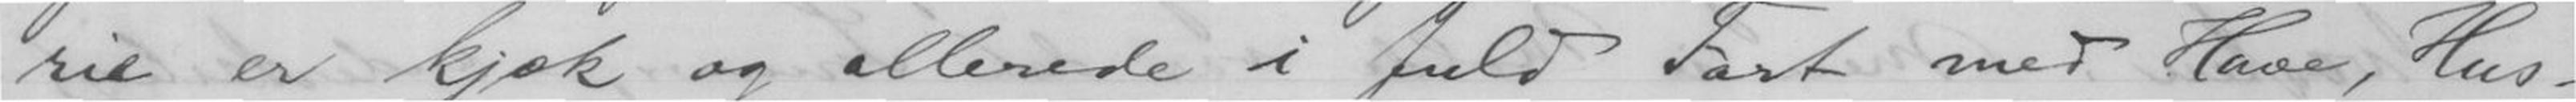rie er kjæk og allerede i fuld Fart med Have, Hus-
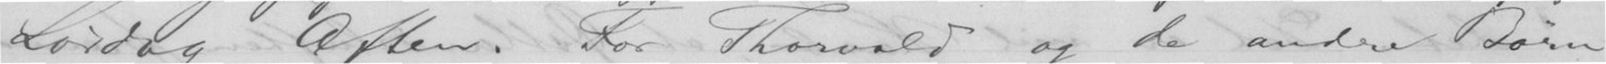Lørdag Aften. For Thorvald og de andre Børn
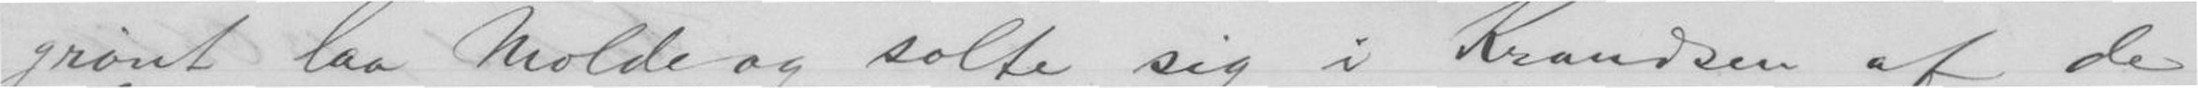grønt laa Molde og solte sig i Krandsen af de
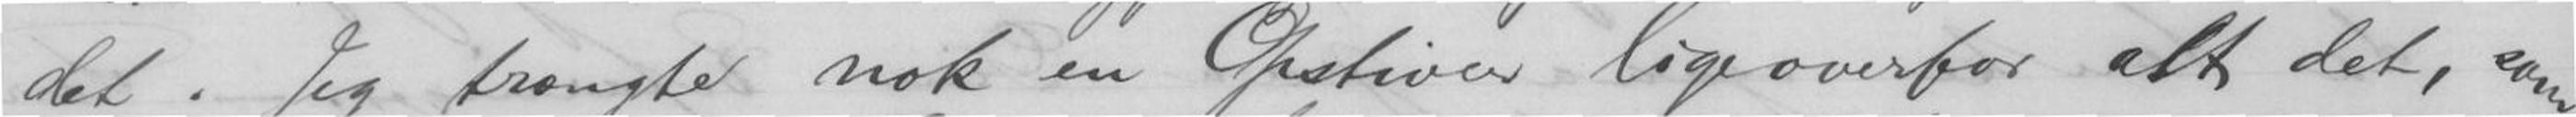det. Jeg trængte nok en Opstiver ligeoverfor alt det, som
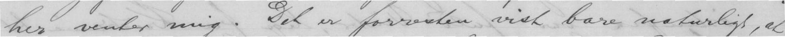her venter mig. Det er forresten vist bare naturligt, at
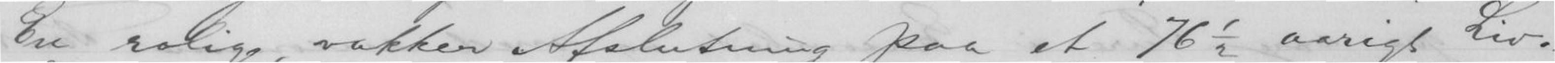En rolig, vakker Afslutning paa et 76½ aarigt Liv.
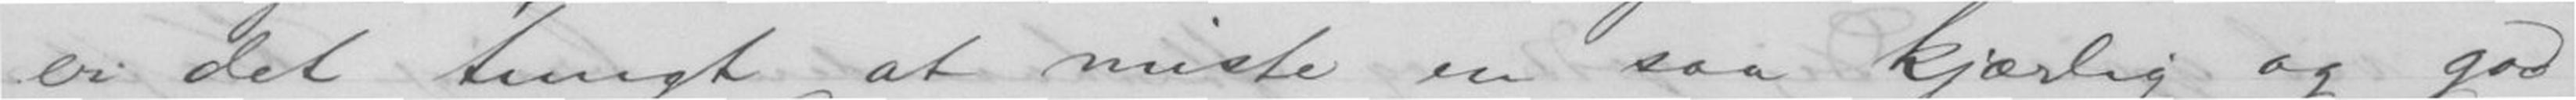er det tungt at miste en saa kjærlig og god
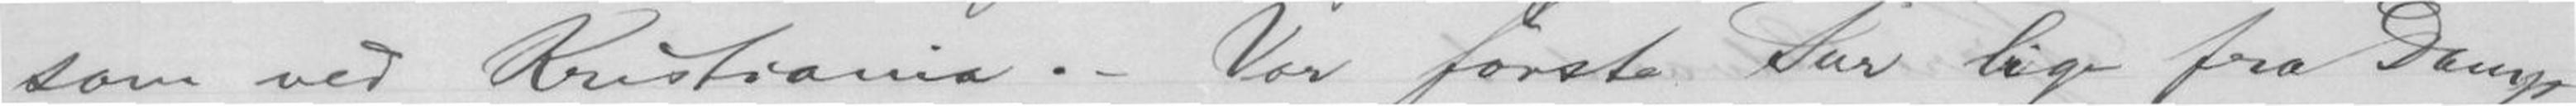som ved Kristiania. - Vor første Tur lige fra Damp-
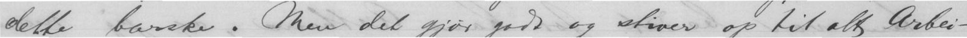dette barske. Men det gjør godt og stiver op til alt Arbei-
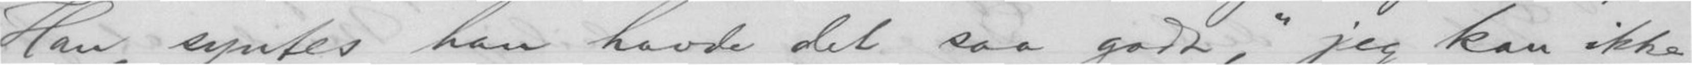Han syntes han havde det saa godt, "jeg kan ikke
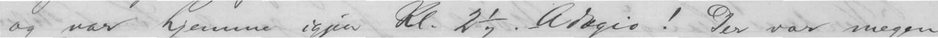og var hjemme igjen Kl. 2½. Adagio! Der var megen
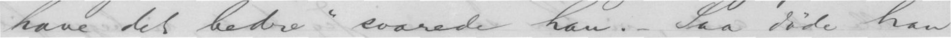have det bedre" svarede han. - Saa døde han
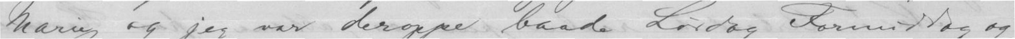Marie og jeg var deroppe baade Lørdag Formiddag og
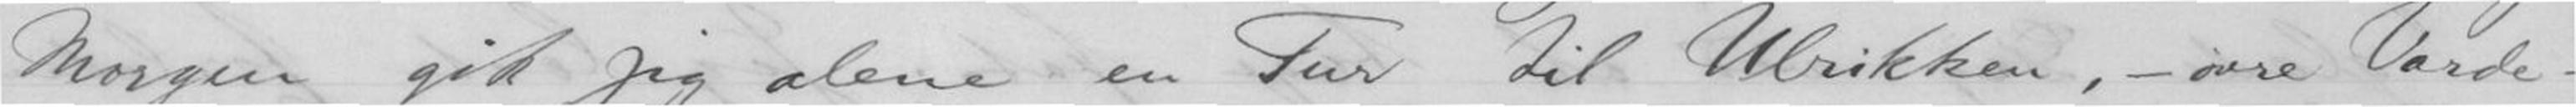Morgen gik jeg Alene en Tur til Ulrikken, - øvre Varde -
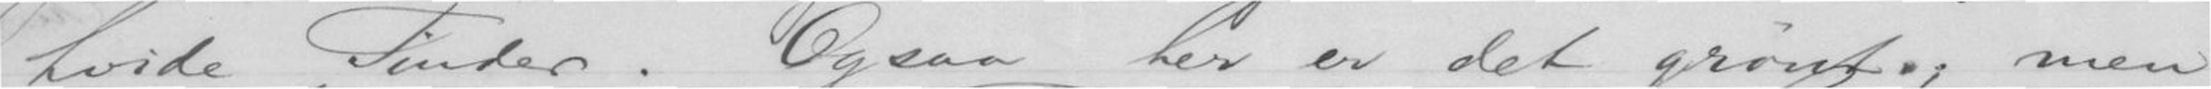hvide Tinder. Ogsaa her er det grønt, men
